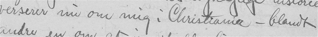verserer nu om mig i Christiania - blandt
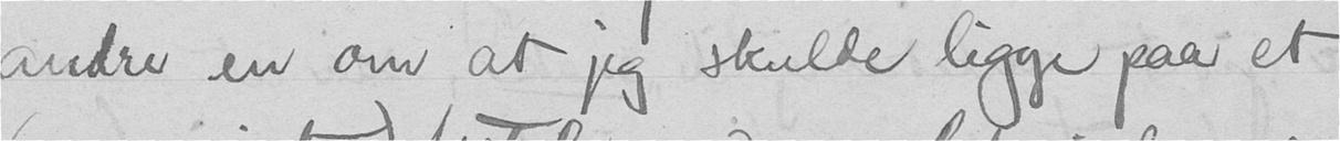andre en om at jeg skulde ligge paa et
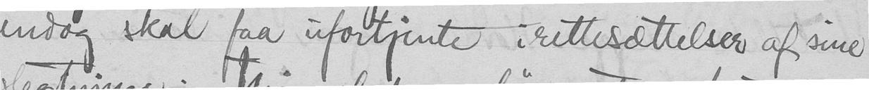endog skal faa ufortjente irettesættelser af sine
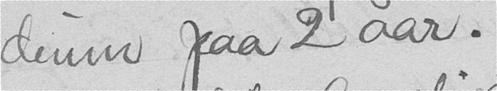dium paa 2 aar.
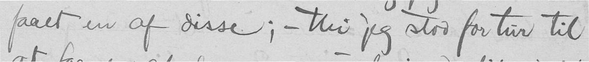faaet en af disse, - thi jeg stod for tur til
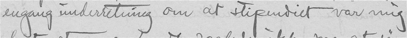engang underretning om at stipendiet var mig
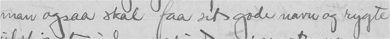man ogsaa skal faa sit gode navn og rygte
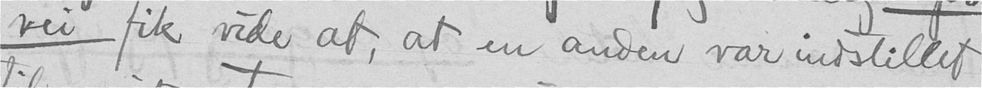vei fik vide at, at en anden var indstillet
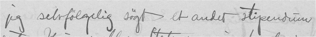jeg selvfølgelig søgt et andet stipendum
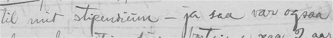til mit stipendium - ja, saa var ogsaa
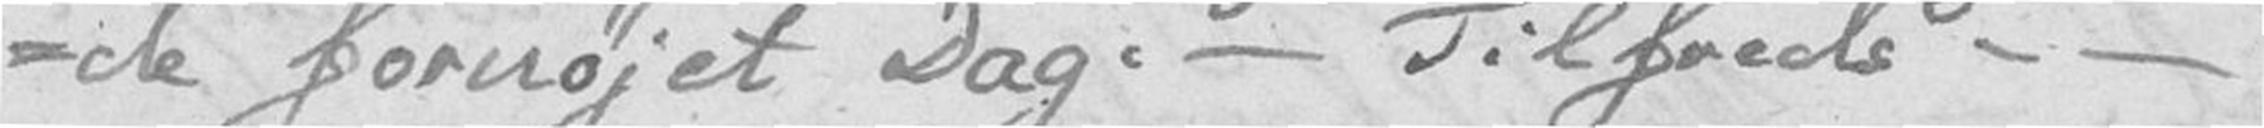-de fornøjet Dag. – Tilfreds – –
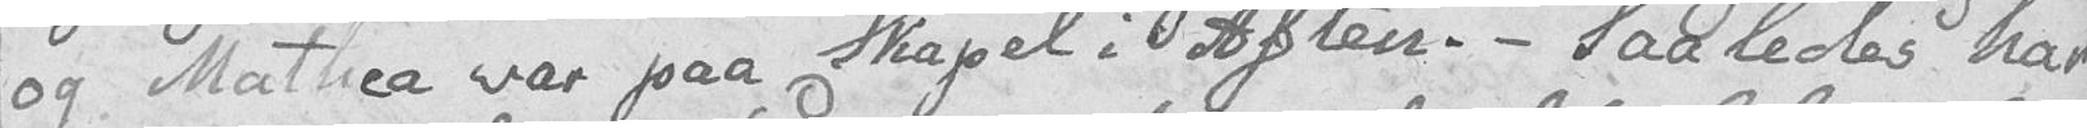og Mathea var paa Skapel i Aften. – Saaledes har
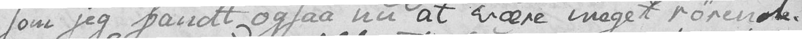som jeg fandt ogsaa nu at være meget rørende.
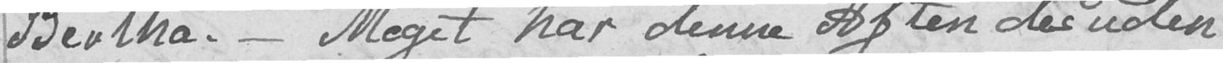Bertha. – Meget har denne Aften desuden
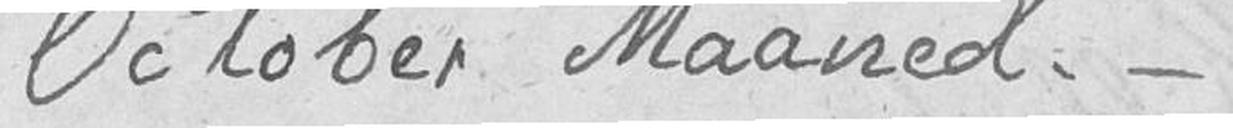October Maaned. –
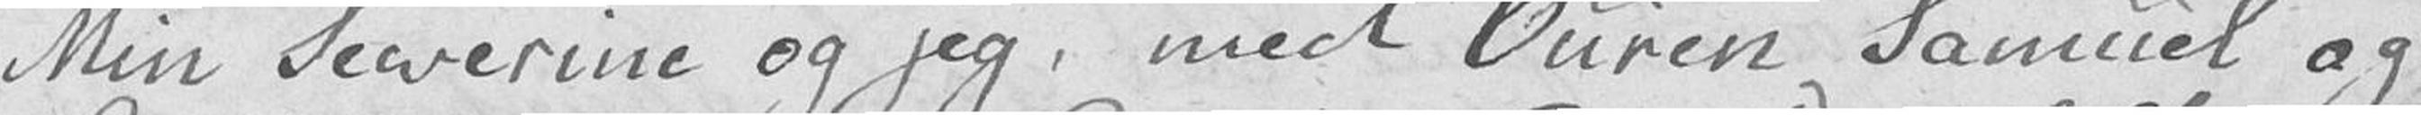Min Severine og jeg, med Ouren Samuel og
